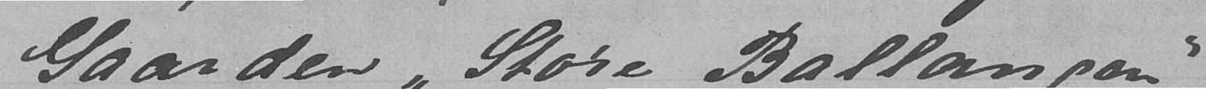Gaarden "Store Ballangen"
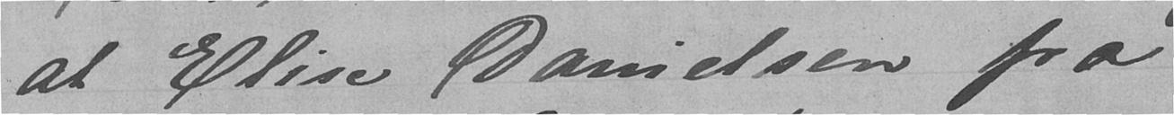at Elise Danielsen fra
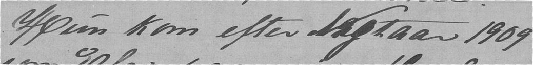Hun kom efter Nytaar 1909
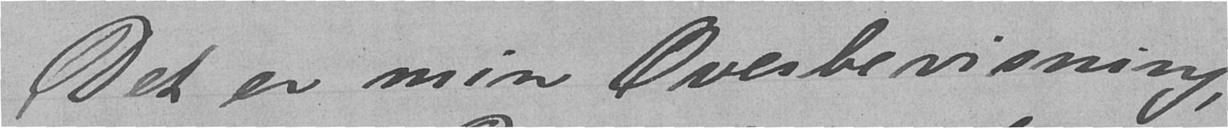Det er min Overbevisning,
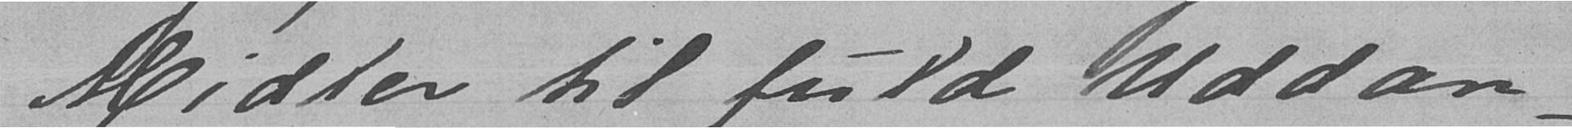Midler til fuld Uddan-
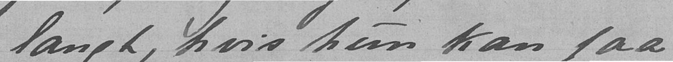langt, hvis hun kan faa
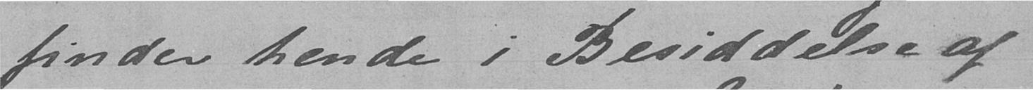finder hende i Besiddelse af
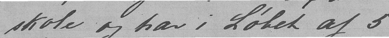skole og har i Løbet af 5
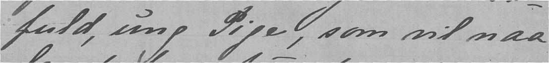fuld, ung Pige, som vil naa
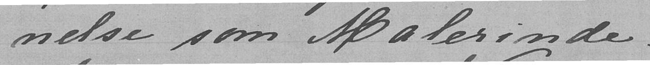nelse som Malerinde.
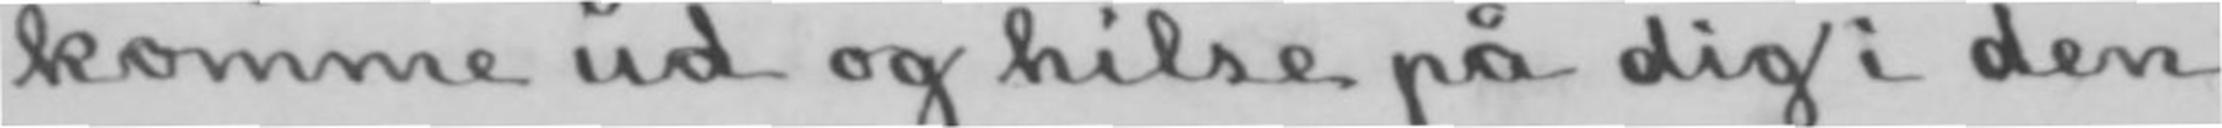komme ud og hilse på dig i den
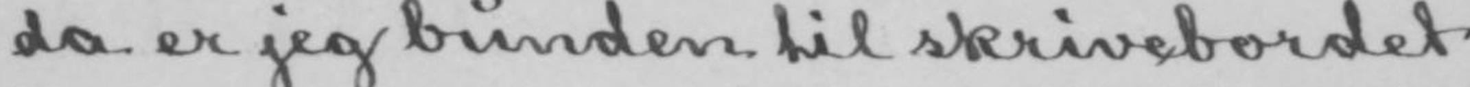da er jeg bunden til skrivebordet
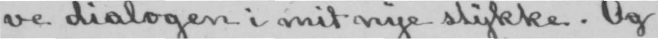ve dialogen i mit nye stykke. Og
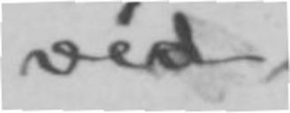véd.
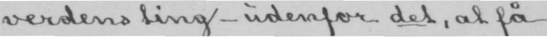verdens ting- udenfor det, at få
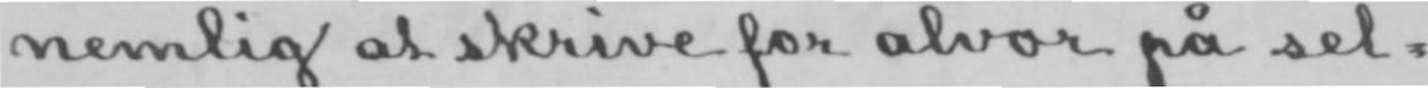nemlig at skrive for alvor på sel-
